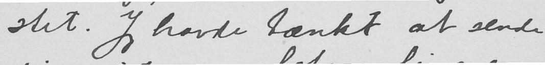stet. Jeg havde tænkt at sende
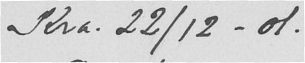Kra. 22/12 - 01.
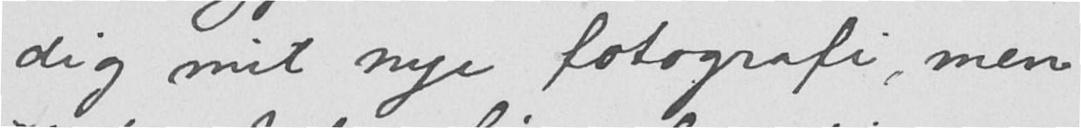dig mit nye fotografi, men
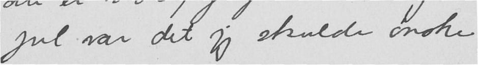jul var det jeg skulde ønske
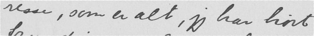resse, som er alt, jeg har hørt
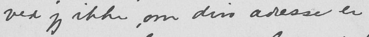ved jeg ikke om din adresse er
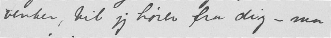venter, til jeg hører fra dig - men
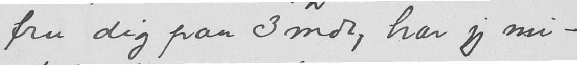fra dig paa 3 mdr, har jeg mi-
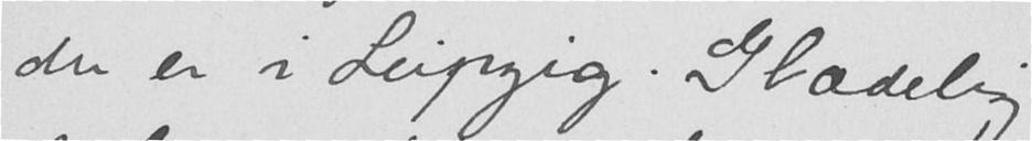du er i Leipzig. Glædelig
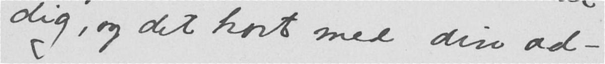dig, og det kort med din ad-
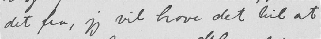det fra, jeg vil have det til at
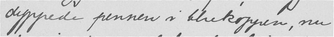dyppede pennen i thekoppen, nu
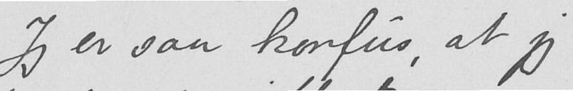Jeg er saa konfus, at jeg
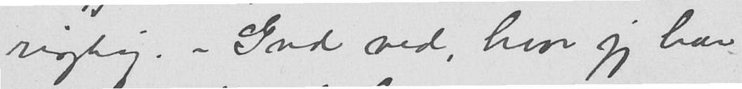rigtig. - Gud ved, hvor  jeg har
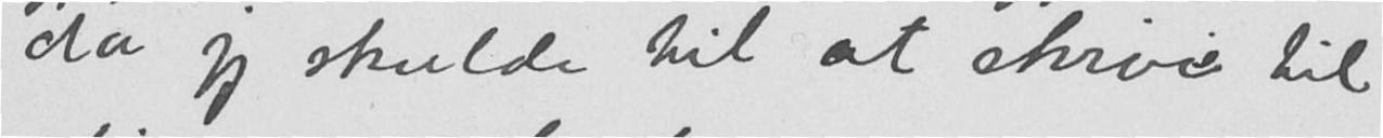da jeg skulde til at skrive til
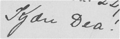Kjære Dea!
